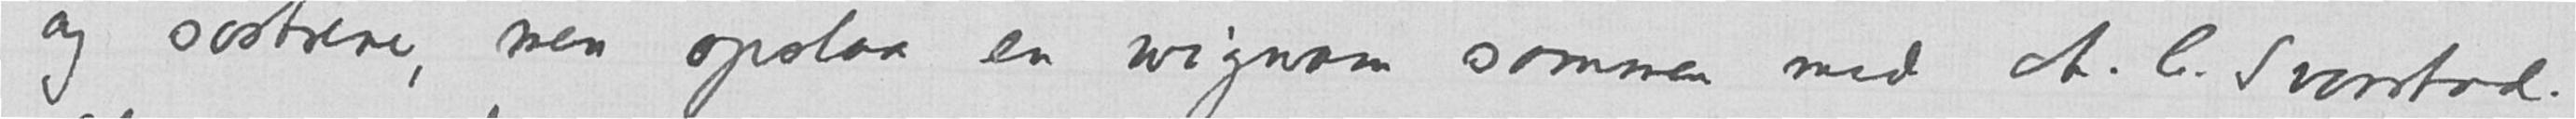og søstrene, men opslaa en wigwam sammen med A.C. Svarstad.
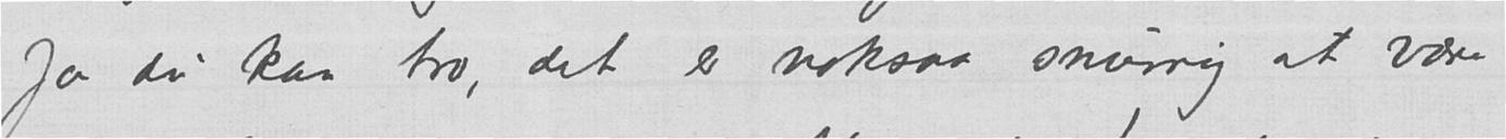Ja du kan tro, det er noksaa snurrig at være
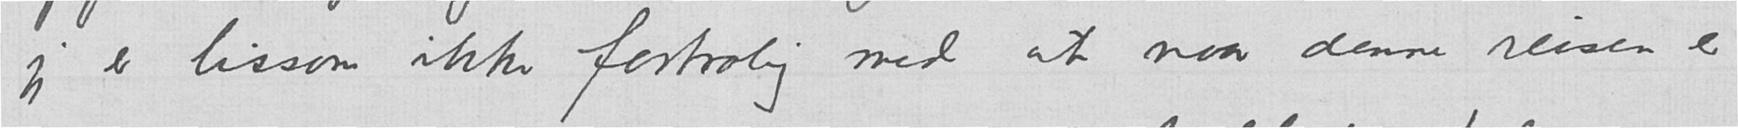jeg er lissom ikke fortrolig med at naar denne reisen er
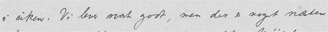i uken. Vi lever svært godt, men der er noget næsten
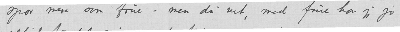spor mere som frue – men du vet, med frue har jeg jo
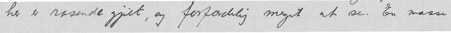her er rasende gjilt, og forfærdelig meget at se. En masse
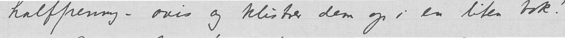halfpenny-avis og klistrer dem op i en liten bok!
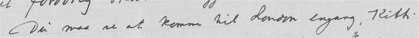Du maa se at komme til London engang, Kitti -
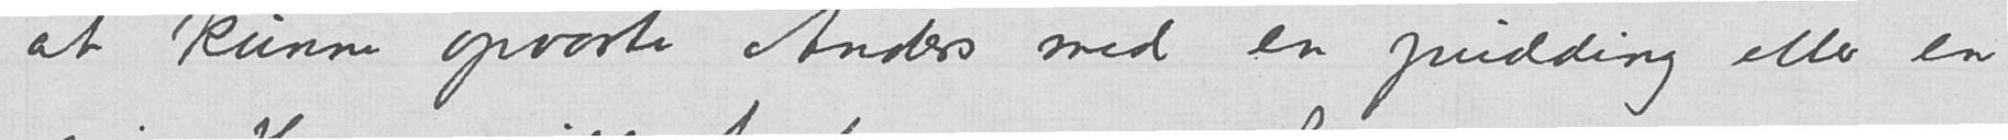at kunne opvarte Anders med en pudding eller en
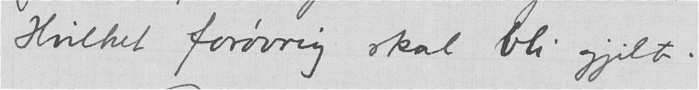Hvilket forøvrig skal bli gjilt.
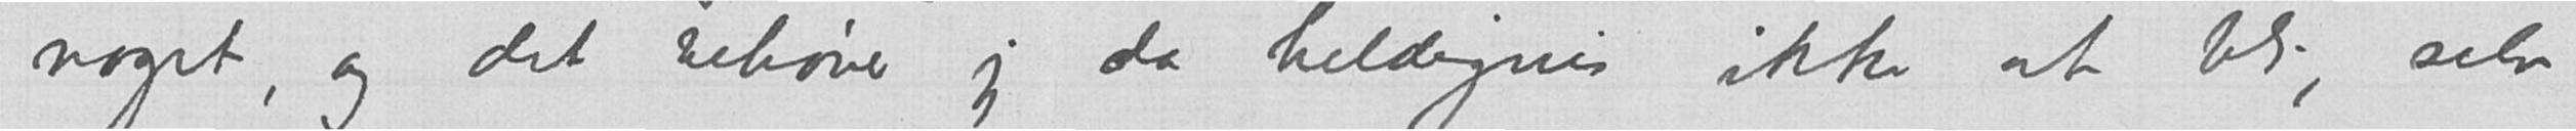noget, og det behøver jeg da heldigvis ikke at bli, selv
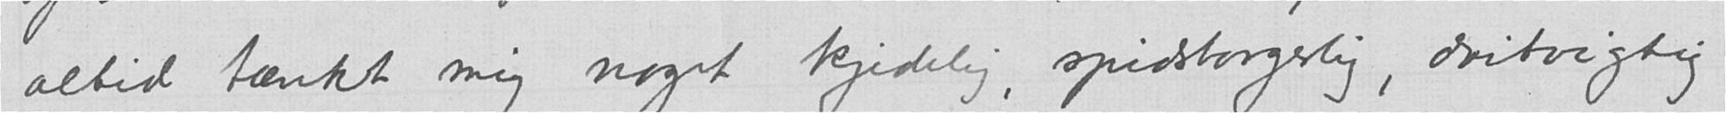altid tænkt mig noget kjedelig, spidsborgerlig, dritvigtig
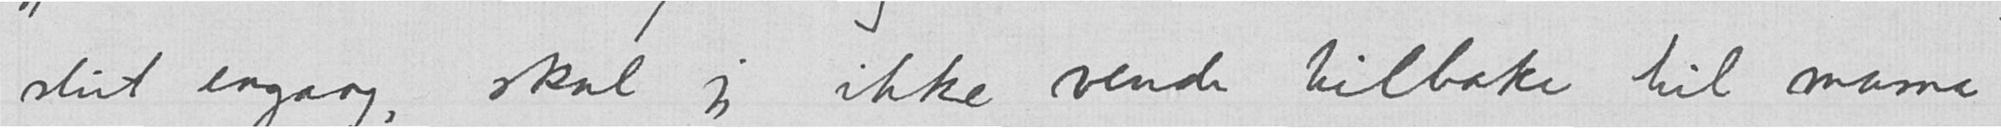slut engang, skal jeg ikke vende tilbake til mama
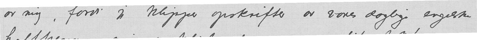av mig, fordi jeg klipper opskrifter av vores daglige engelske
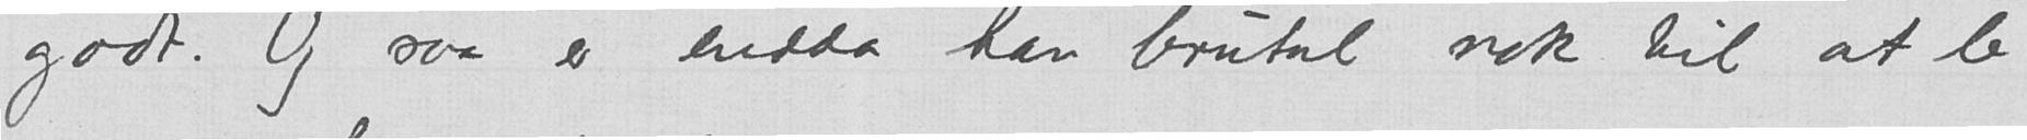godt. Og saa er endda han brutal nok til at le
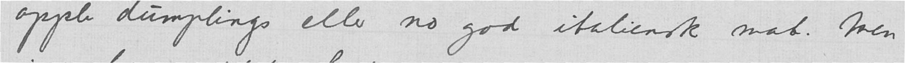apple dumplings eller no god italiensk mat. Men
